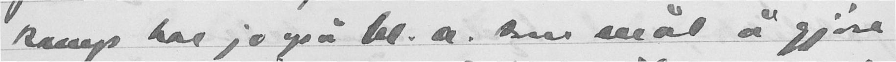kamp har jo også bl. a, som mål å gjøre
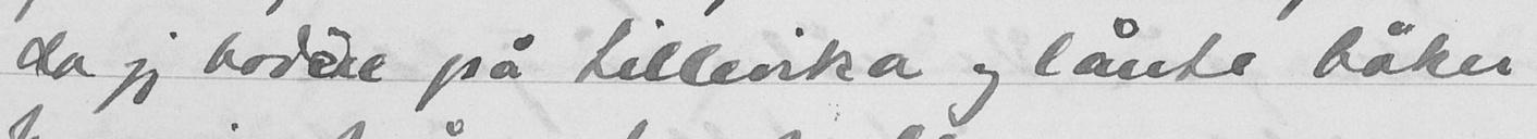da jeg bodde på Lillivika og lånte bøker
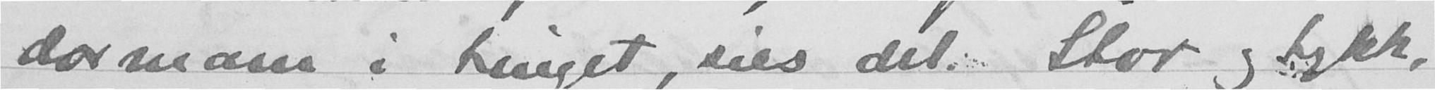dormann i tinget, sies det. Stor og tykk,
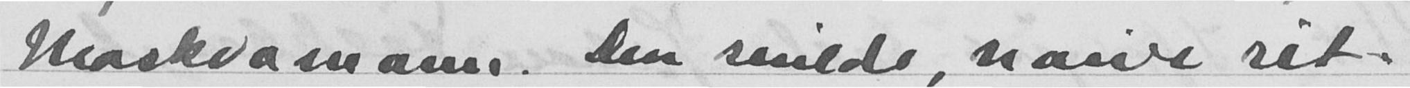Maskvamanig. Den snilde, naive rit-
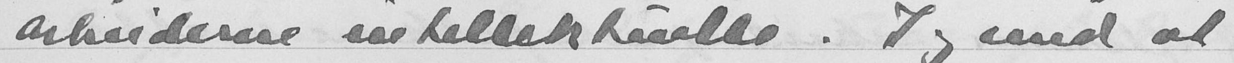arbeiderne intellektuelle. I og med at
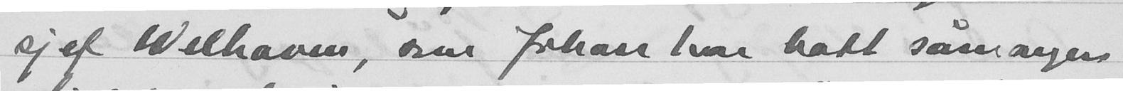sjef Welhaven, som Johan har hatt såmangen
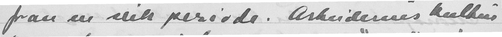foran en slik periode. Arbeidernes kultur
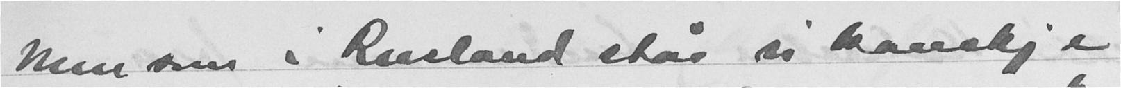Men som i Rusland står vi kanskje
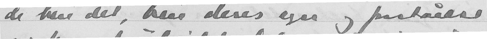de blir det, blir deres egen og forståelse
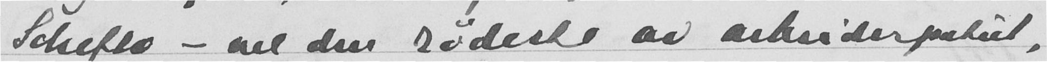Scheflo - vel den rødeste av arbeiderpartiet,
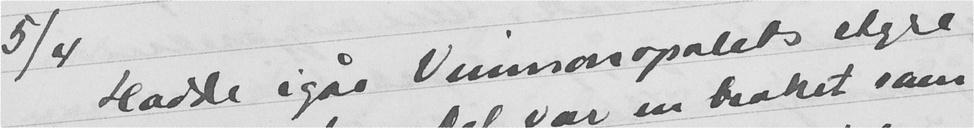5/4 Hadde igår Vinmonopolets elgre
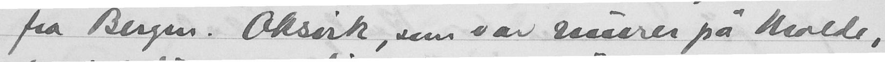fra Bergen. Akseik, som var murer på Molde,
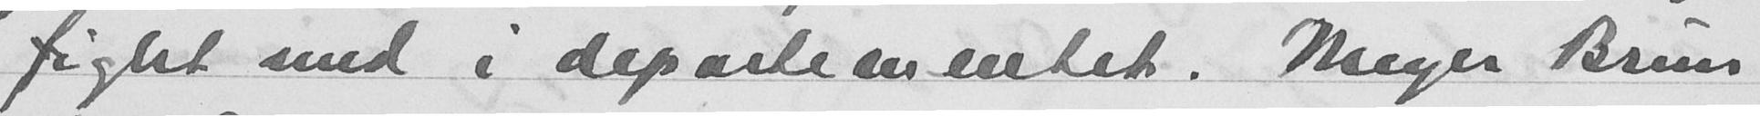fight med i departementet. Meyer Brins
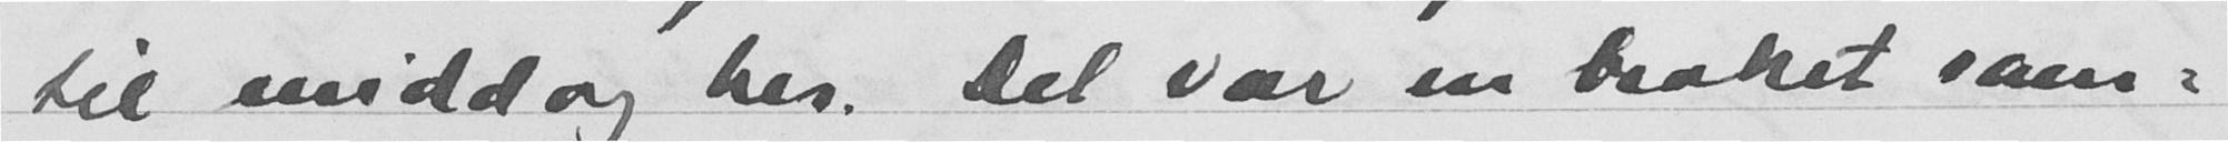til middag her. Det var en broket sam-
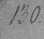130.
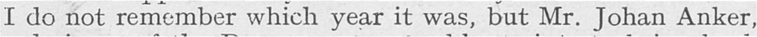I do not remember which year it was, but Mr. Johan Anker,
